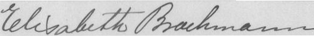Elisabeth Brochmann
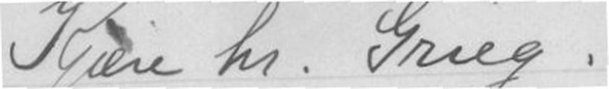Kjære hr. Grieg.
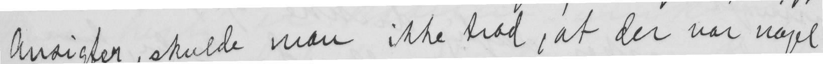Ansigter, skulde man ikke trod, at der var nogel
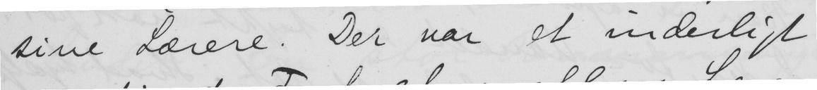sine Lærere. Der var et inderligt
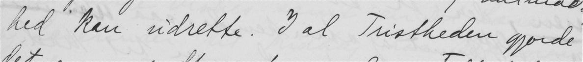hed kan udrette. I al Tristheden gjorde
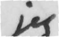jeg
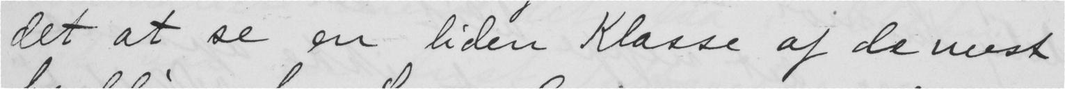det at se en liden Klasse af de mest
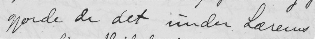gjorde de det under Lærerns
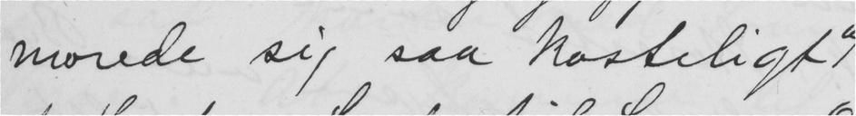morede sig saa kosteligt
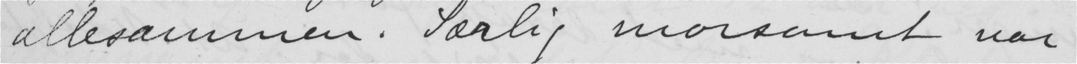allesammen. Særlig morsomt var
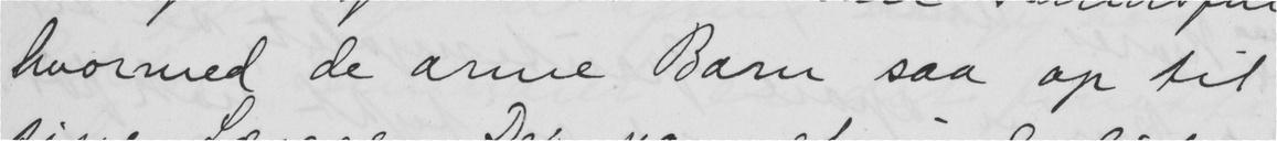hvormed de arme Barn saa op til
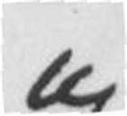og
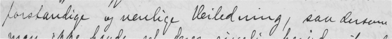forstandige og venlige Veiledning, saa dersom
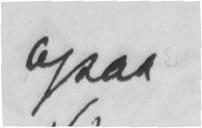ogsaa
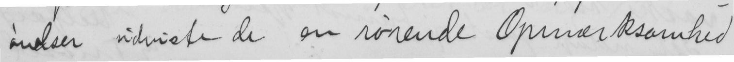øvelser udviste de en rørende Opmærksomhed
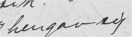hengav sig
